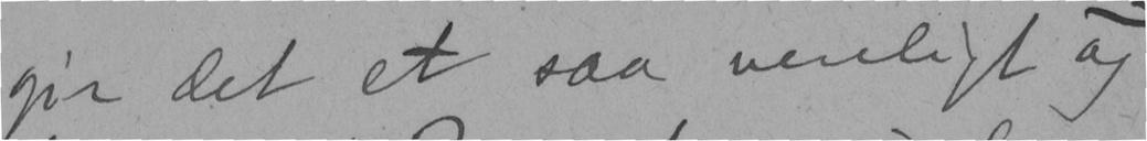gir det et saa venligt og
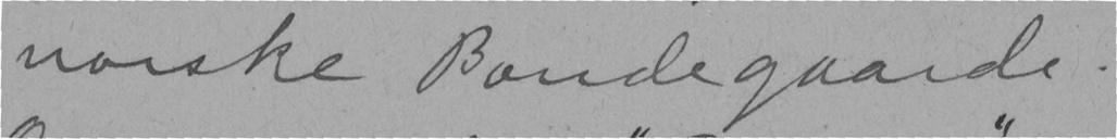norske Bondegaarde.
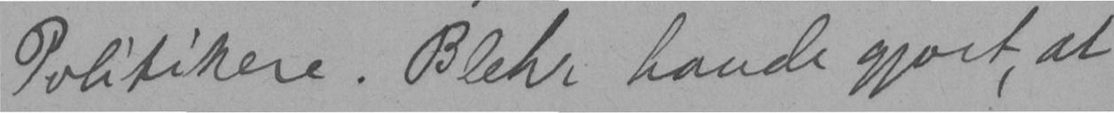Politikere. Blehr havde gjort, at
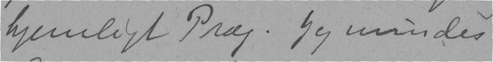hjemligt Præg. Jeg mindes
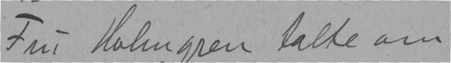Fru Holmgren talte om
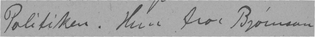Politiken. Hun tror Bjørnson
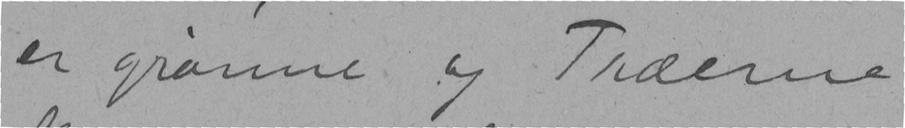er grønne og Træerne
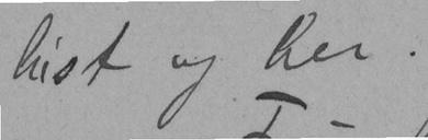hist og her.
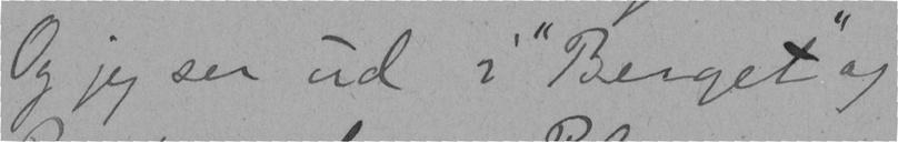Og jeg ser ud i "Berget" og
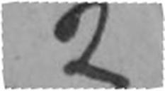2
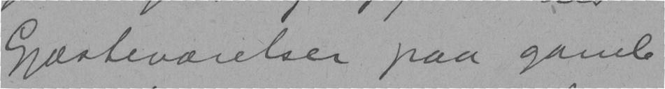Gjæsteværelser paa gamle
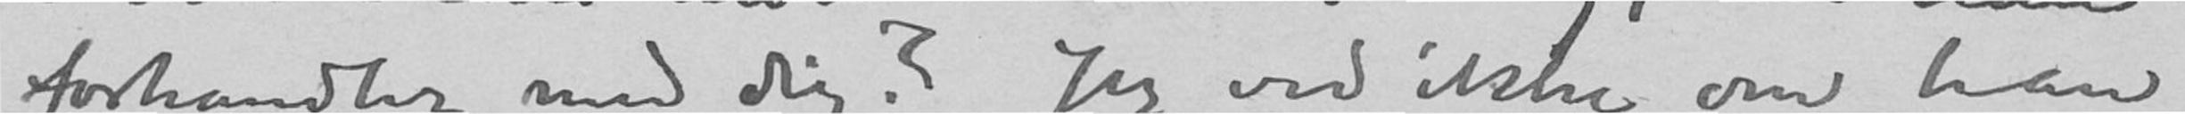forhandler med dig? Jeg ved ikke om han
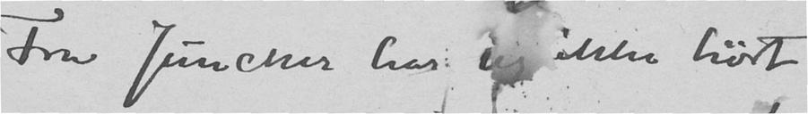Fra Juncker har jeg ikke hørt
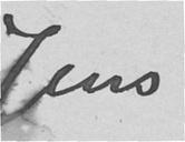Jens
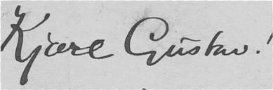Kjære Gustav!
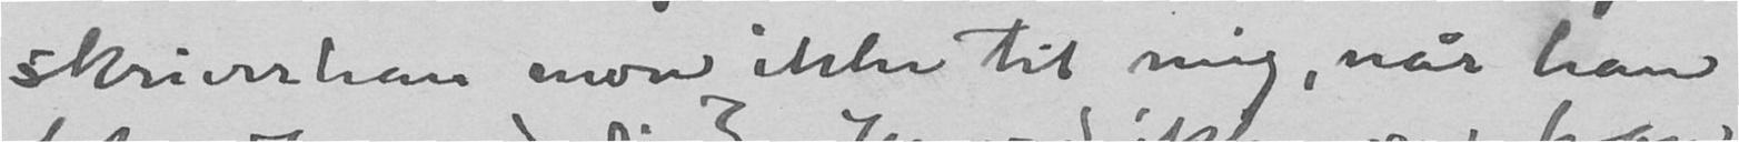skriver han mon ikke til mig, når han
